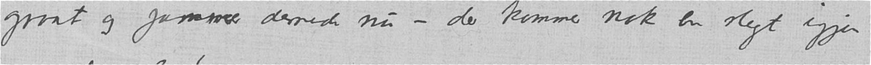graat og jammer dernede nu – der kommer nok en slegt igjen
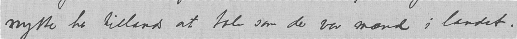nytte her tillands at tale som der var mænd i landet.
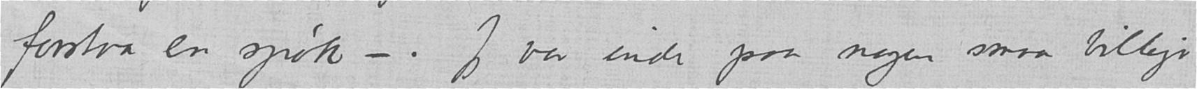forstaa en spøk –. Jeg var inde paa nogen smaa billige
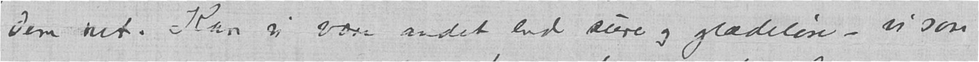Dem ret. Kan vi være andet end sure og glædeløse - vi som
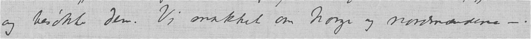og besøkte Dem. Vi snakket om Norge og nordmændene –.
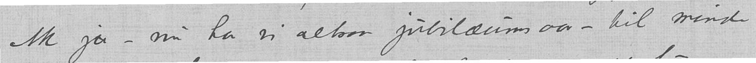Ak ja – nu har vi altsaa jubilæumsaar – til minde
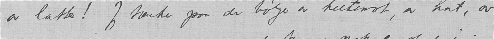av latter! Jeg tænker paa de bølger av heltemot, av hat, av
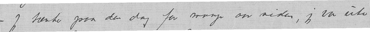– Jeg tænker paa den dag for mange aar siden, jeg var ute
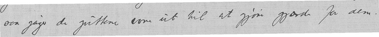saa jager de guttene vore ut til at gjøre gjærde for dem.
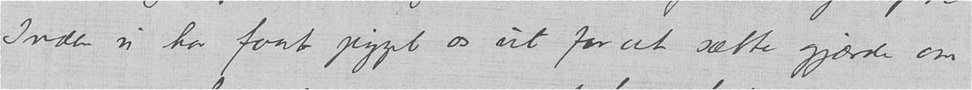Inden vi har faat pigget os ut for at sætte gjærde om
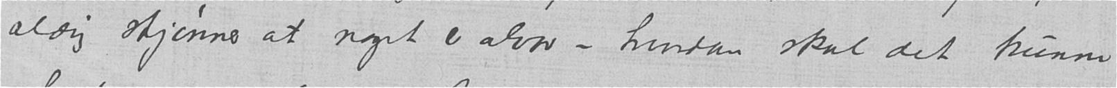aldrig skjønner at noget er alvor – hvordan skal det kunne
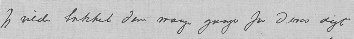Jeg vilde takket Dem mange ganger for Deres digt
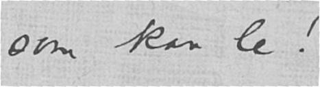som kan le!
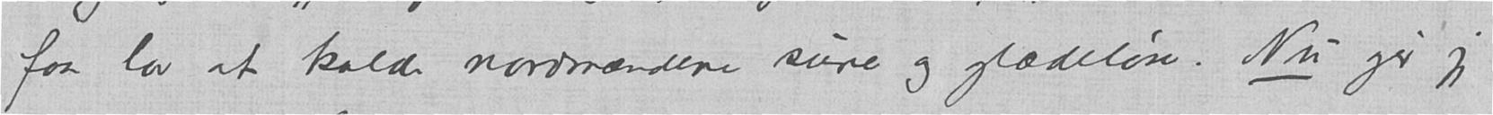faa lov at kalde nordmændene sure og glædeløse. Nu gir jeg
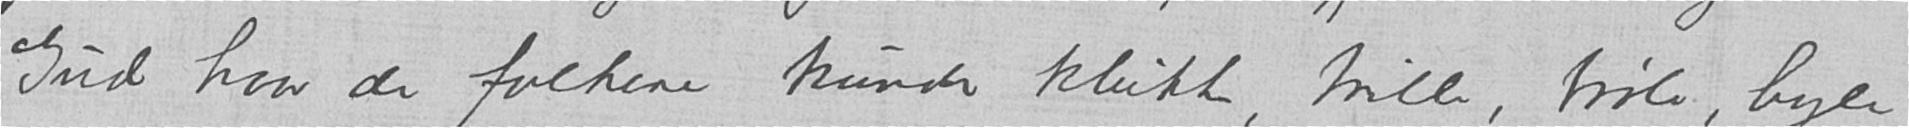Gud hvor de folkene kunde klukke, trille, brøle, hyle
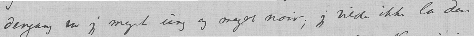Dengang var jeg meget ung og meget naiv -, jeg vilde ikke la Dem
